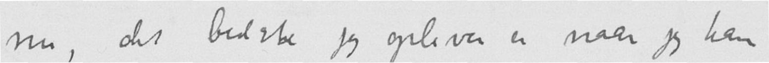nu, det bedste jeg oplever er naar jeg kan
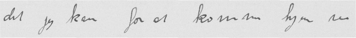det jeg kan for at komme hjem saa
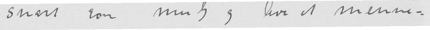snart som mulig og leve et menne-
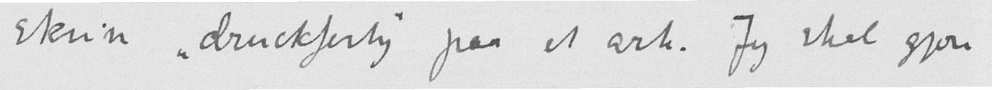skrive "druckfertig" paa et ark. Jeg skal gjøre
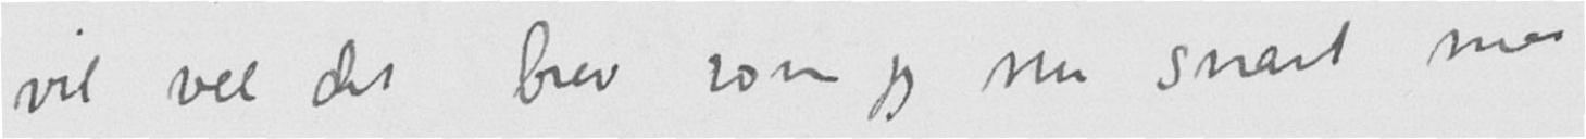vil vel det brev som jeg nu snart maa
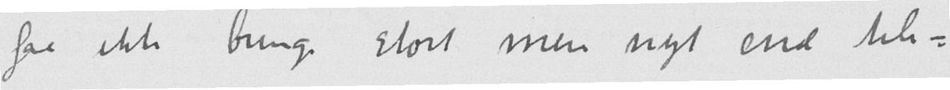faa ikke bringe stort mere nyt end tele-
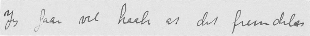Jeg faar vel haabe at det fremdeles
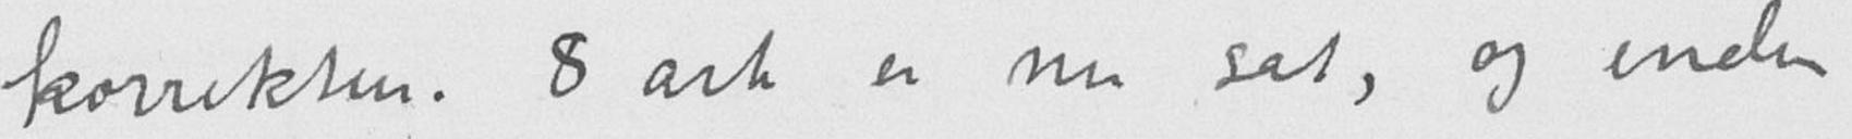korrektur. 8 ark er nu sat, og inden
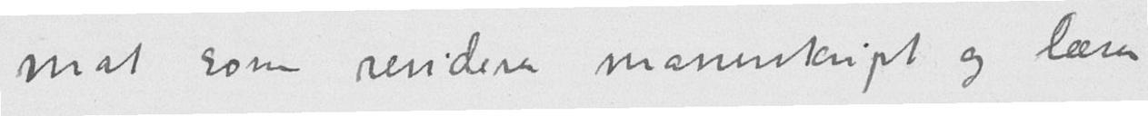mat som reviderer manuskript og læser
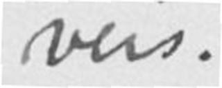veis.
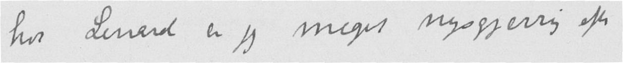hos Lenard er jeg meget nysgjerrig efter
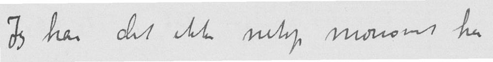Jeg har det ikke netop morsomt her
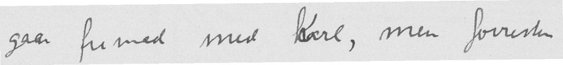gaar fremad med Karl, men forresten
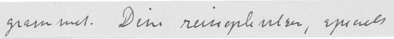grammet. Dine reiseoplevelser, specielt
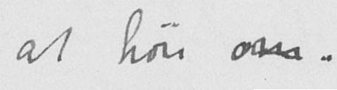at høre om.
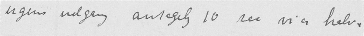ugens udgang antagelig 10 saa vi er halv-
